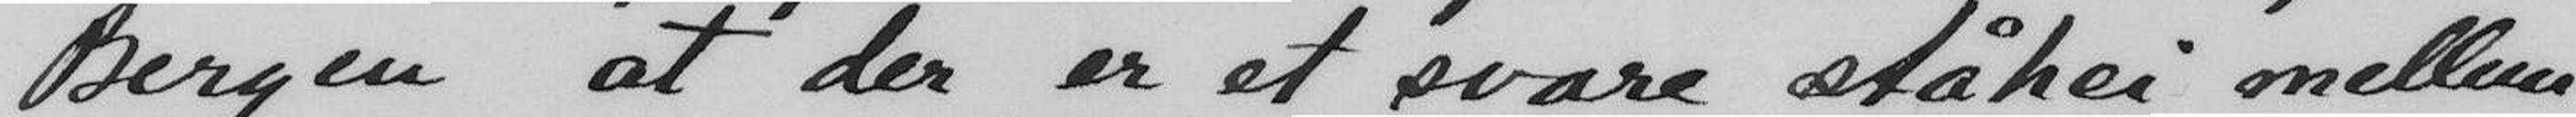Bergen at der er et svare ståhei mellem
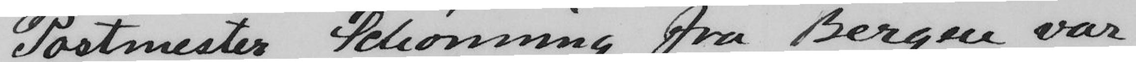Postmester Schønning fra Bergen var
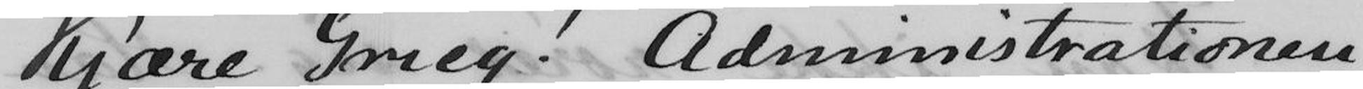Kjære Grieg! Administrationen
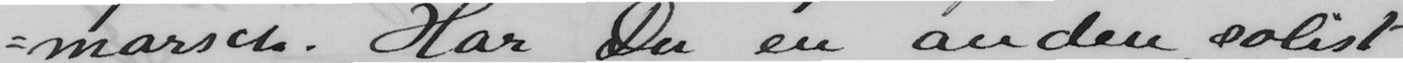marsch. Har du en anden solist
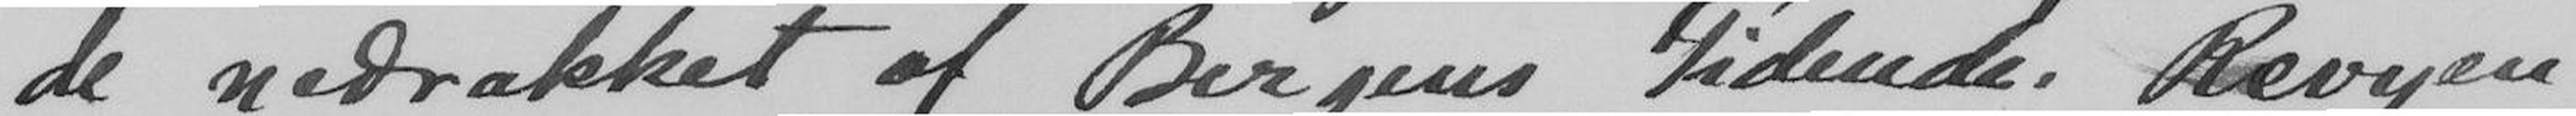de nedrakket af Bergens Tidende. Revyen
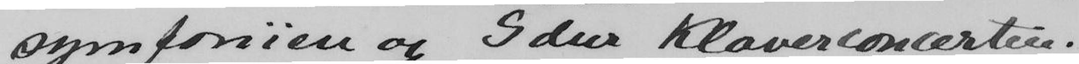symfonïen og G dur Klaverconcerten. --
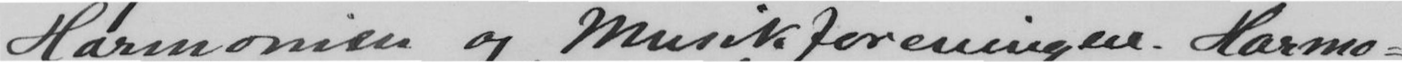Harmonien og Musikforeningen. Harmo-
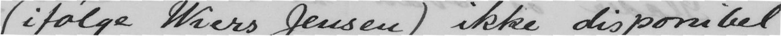(ifølge Wiers Jensen) ikke disponibel
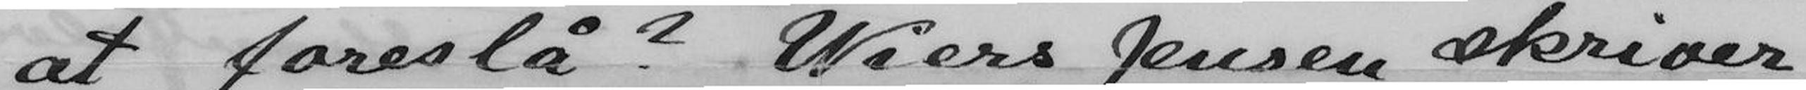at foreslå? Wiers Jensen skriver
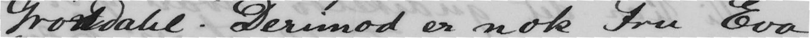Grøndahl. Derimod er nok Fru Eva
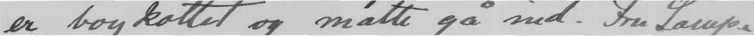er boykottet og måtte gå ind. Fru Lampe
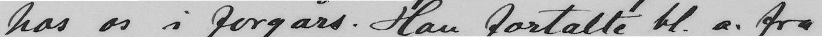hos os i forgårs. Han fortalte bl.a. fra
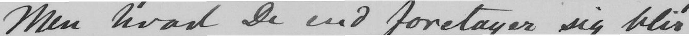Men hvad De end foretager sig blir
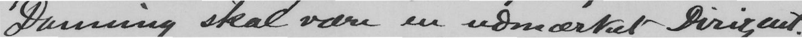Danning skal være en udmærket Dirigent.
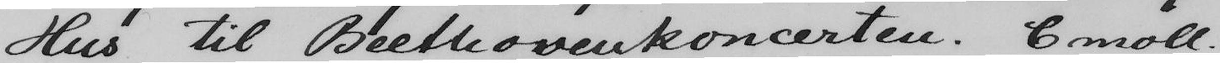Hus til Beethovenkoncerten. C moll
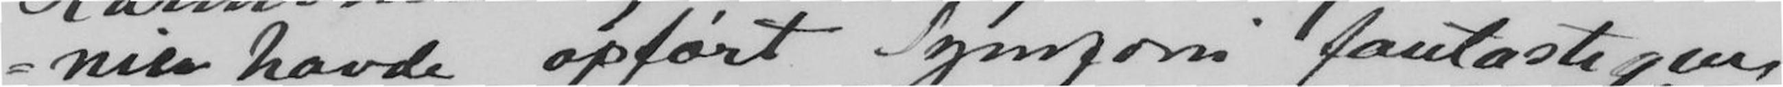nien havde opført Symfoni fantastique
